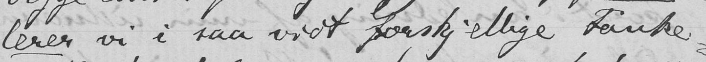lerer vi i saa vidt forskjellige tanke-
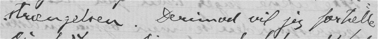strængelsen. Derimod vil jeg fortælle
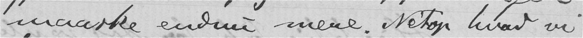maaske endnu mere. Netop hvad vi
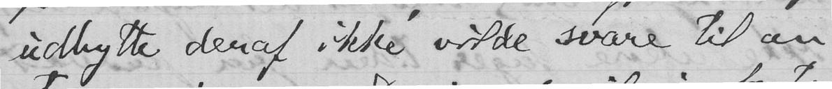udbytte deraf ikke vilde svare til an-
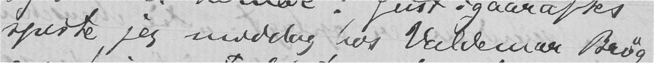spiste jeg middag hos Valdemar Brøg-
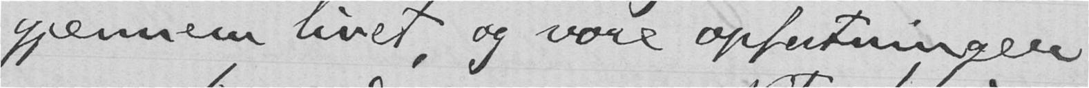gjennem livet, og vore opfatninger
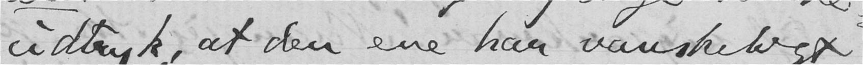udtryk, at den ene har vanskeligt
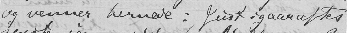og venner hernede: Just igaaraftes
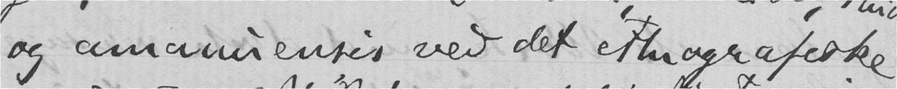og amanuensis ved det ethnografiske
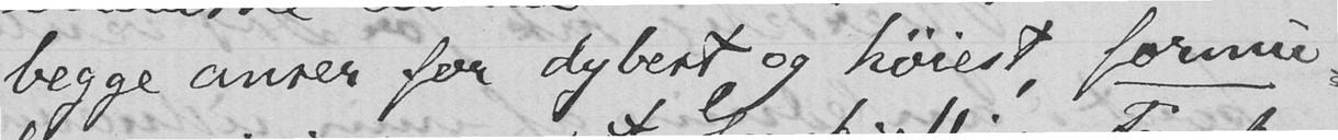begge anser for dybest og høiest, formu-
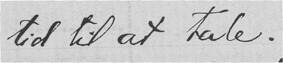tid til at tale.
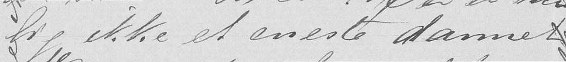lig ikke et eneste dannet
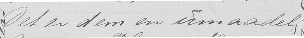Det er dem en umaadelig
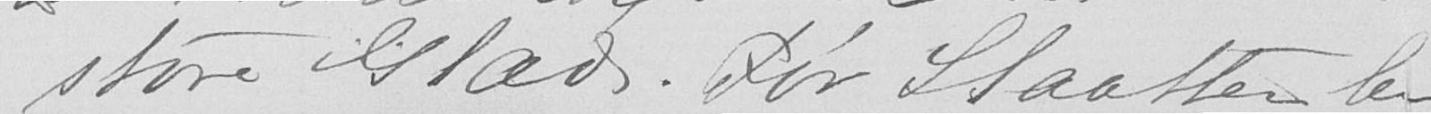store Glæde. Før Slaatten be-
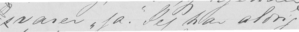svarer "ja". Jeg har aldrig
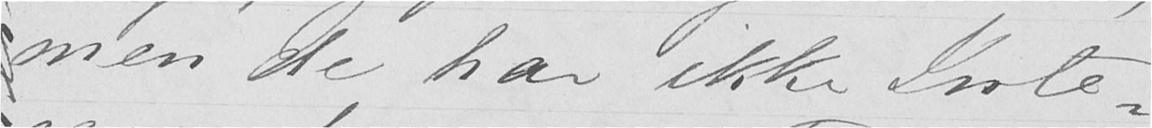men de har ikke Inte-
